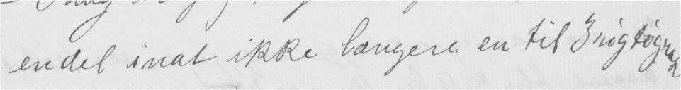en del inat ikke længere en til 3, rigtignok
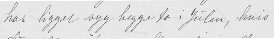har ligget syg begge to i Julen, hvis
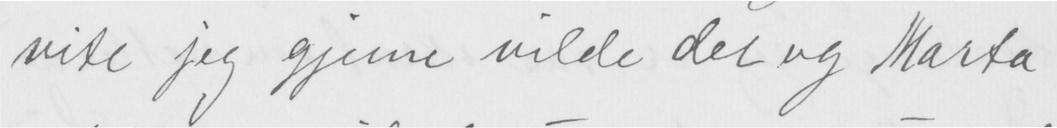vite jeg gjerne vilde det og Marta
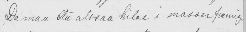Da maa du altsaa hilse i masser fra mig.
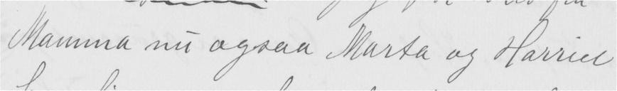Mamma nu ogsaa Marta og Harriet
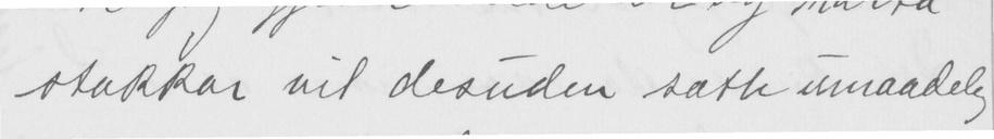stakkar vil desuden sætte umaadelig
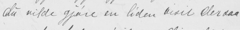du vilde gjøre en liden visit der saa
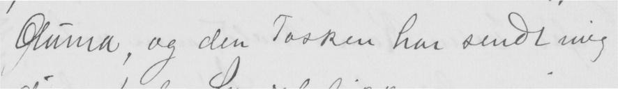Gluma, og den Tosken har sendt mig
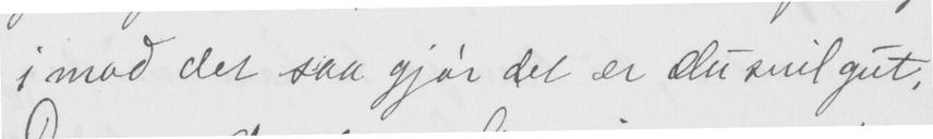i mod det saa gjør det er du snil gut.
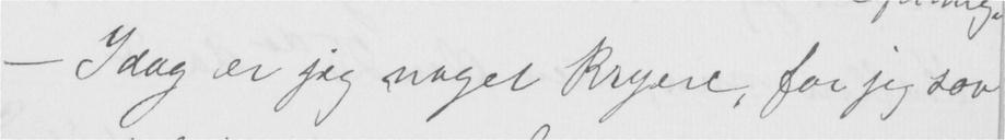– Idag er jeg noget kryere, for jeg sov
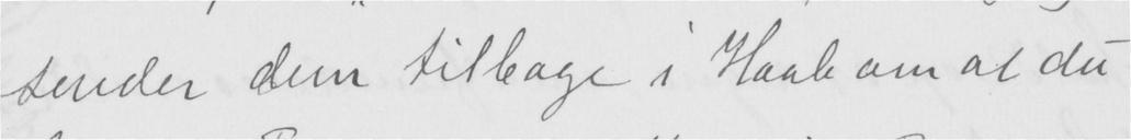sender dem tilbage i Haab om at du
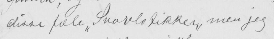disse fæle "Svovelstikker" men jeg
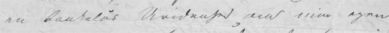en tankeløs Uvidenhed om min egen
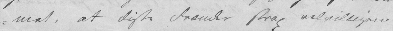met, at disse Frænder strax velvilligen
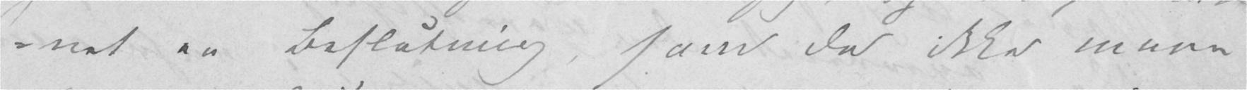net en Beslutning, som De ikke maae
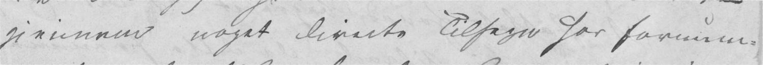gjennem noget directe Tilsagn har fornum-
